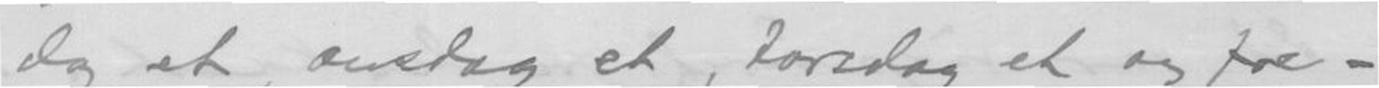dag et, onsdag et, torsdag et og fre-
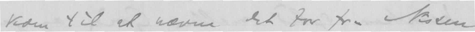kom til at nævne det for fr. Nissen
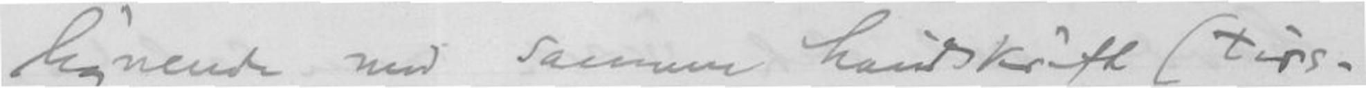lignende med samme håndskrift (tirs-
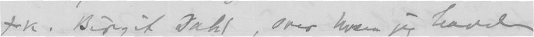frk. Birgit Dahl, om hvem jeg havde
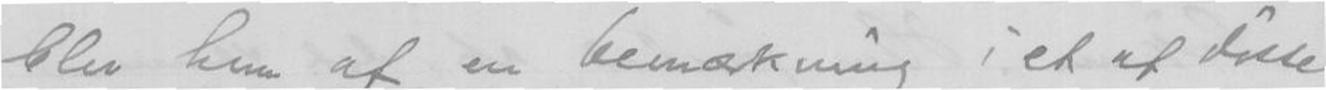blev hun af en bemærkning i et af disse
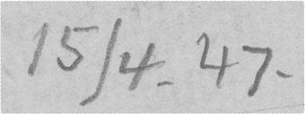15/4. 47.
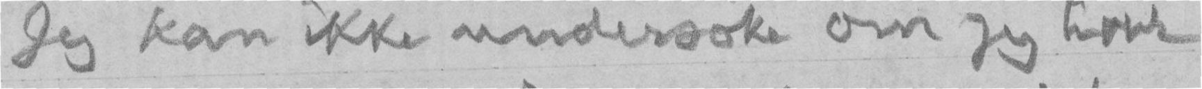Jeg kan ikke undersøke om jeg hadde
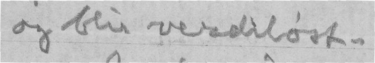og blir verdiløst.
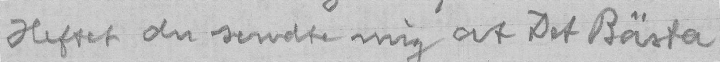Heftet du sendte mig at Det Bästa
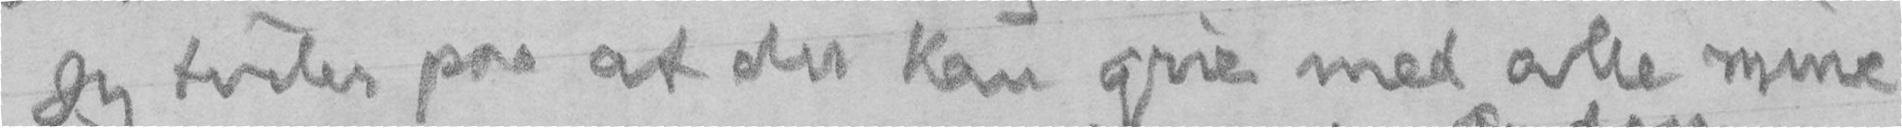Jeg tviler paa at du kan greie med alle mine
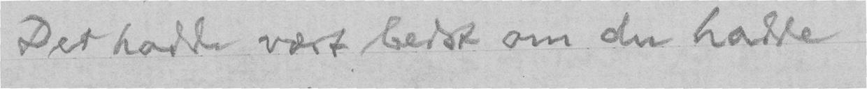Det hadde vært bedst om du hadde
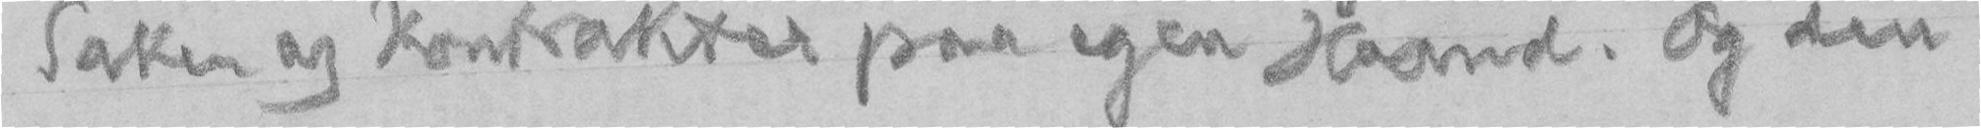Saker og Kontrakter paa egen Haand. Og den
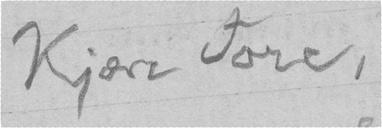Kjære Tore,
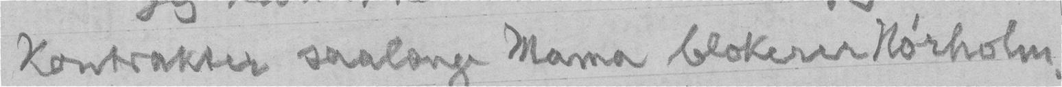Kontrakter saalænge Mama blokerer Nørholm.
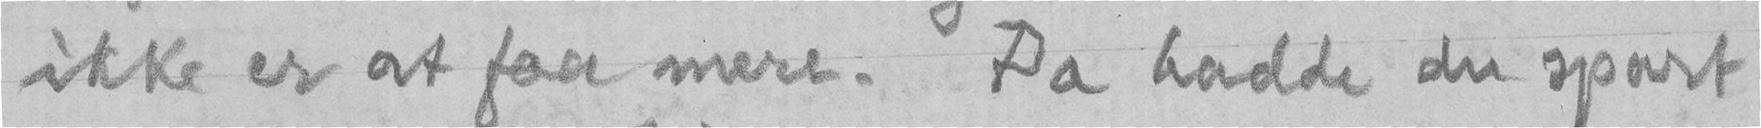ikke er at faa mere. Da hadde du spart
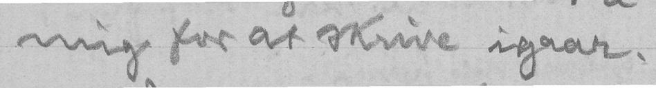mig for at skrive igaar.
